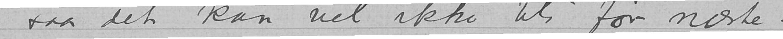saa det kan vel ikke bli før næste
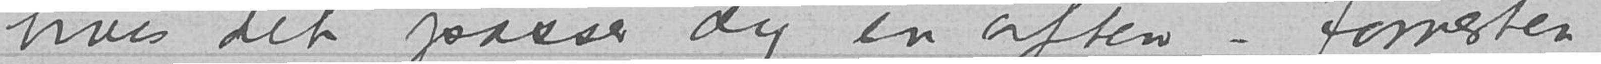hvis det passer dig en aften – forresten
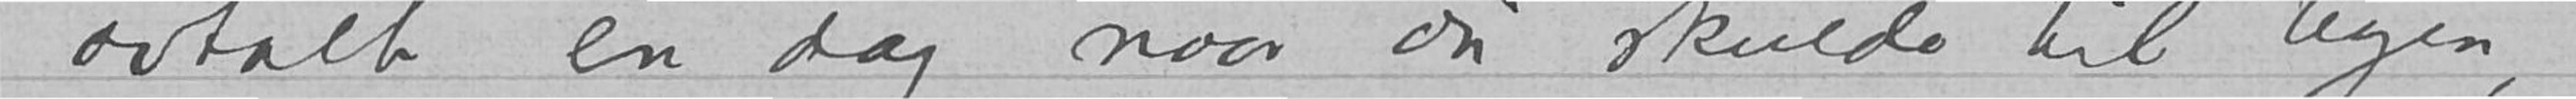avtalt en dag, naar du skulde til byen,
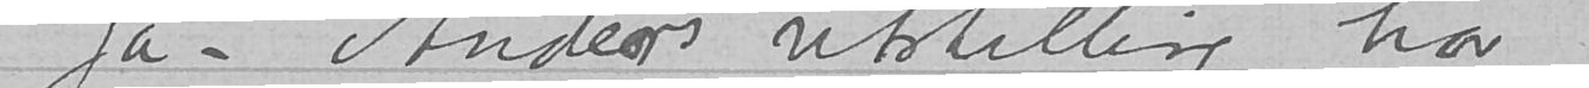Ja – Anders utstilling har
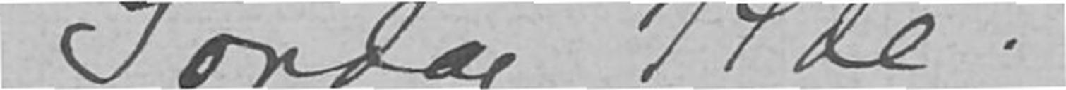Søndag 14de.
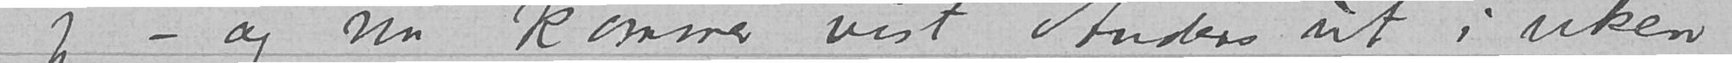jeg - og nu kommer vist Anders ut i uken
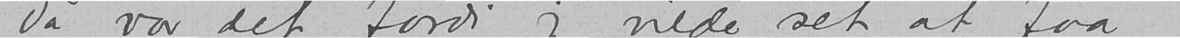Da var det fordi jeg vilde set at faa
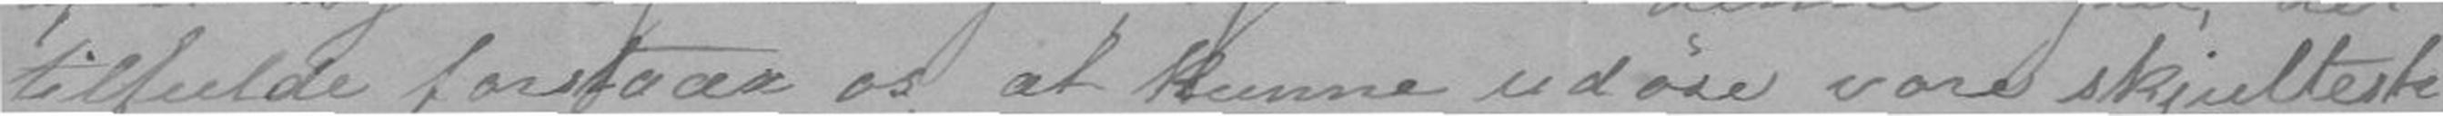tilfulde forstaar os, at kunne udøse vore skjulteste
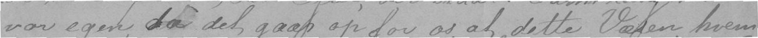vor egen, da det gaar op for os, at dette Væsen, hvem
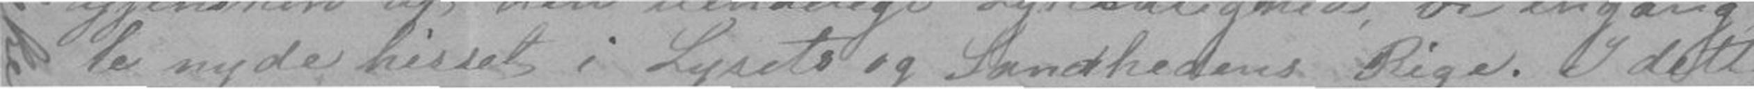le nyde hisset i Lysets og Sandhedens Rige. I dette
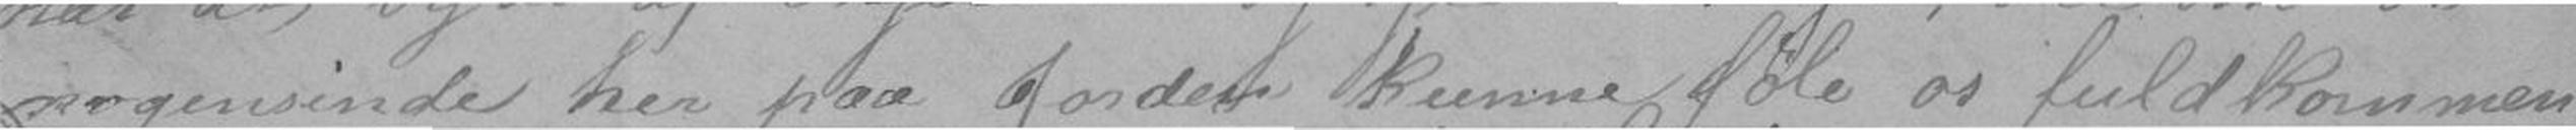nogensinde her paa Jorden kunne føle os fuldkommen
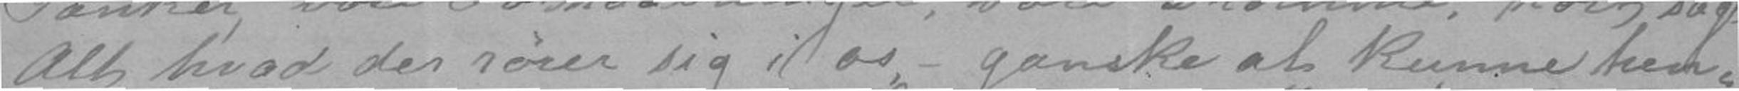Alt hvad der rører sig i os - ganske at kunne hen-
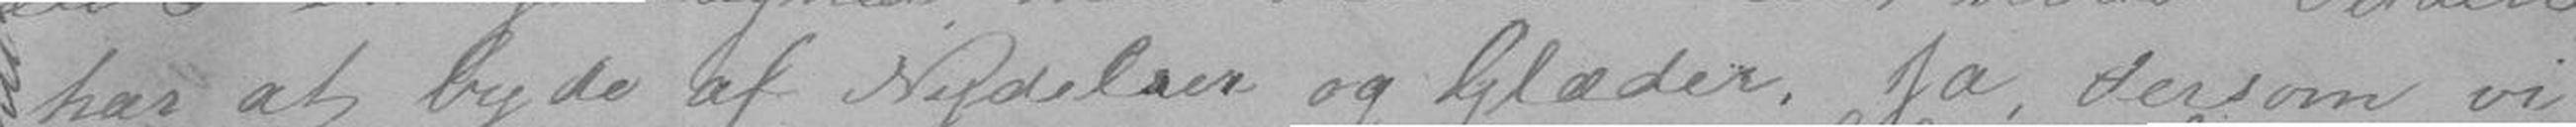har at byde af Nydelser og Glæder, Ja, dersom vi
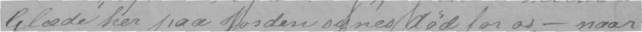Glæde her paa Jorden synes død for os, - naar
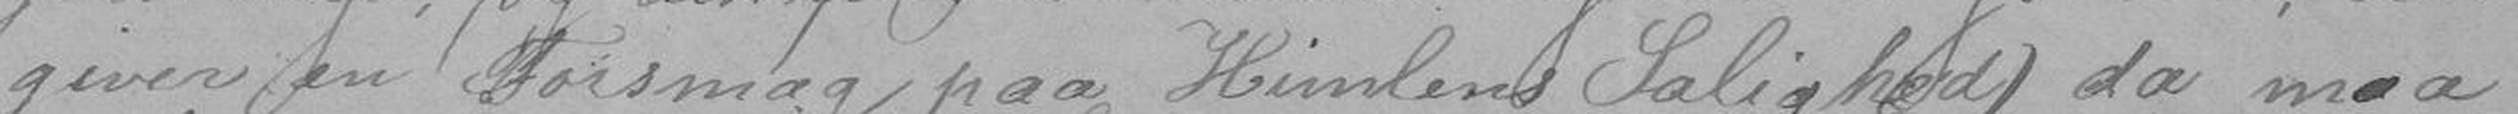giver en Forsmag paa Himlens Salighed) da maa
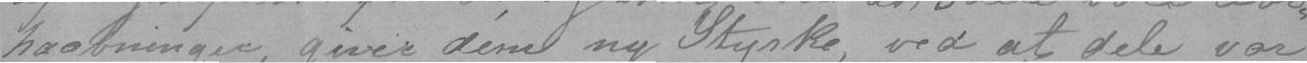haabninger, giver dem ny styrke, ved at dele vor
